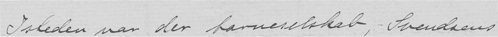Isteden var der barneselskab, – Svendsens
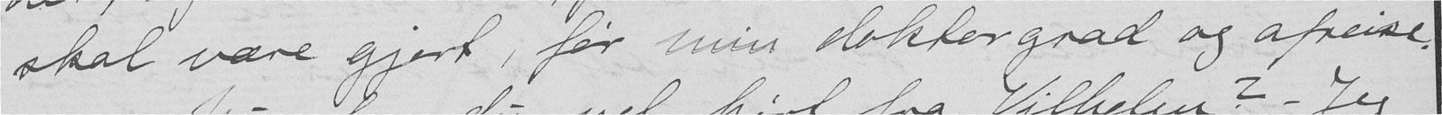skal være gjort, før min doktorgrad og afreise.
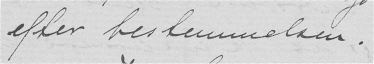efter bestemmelsen.
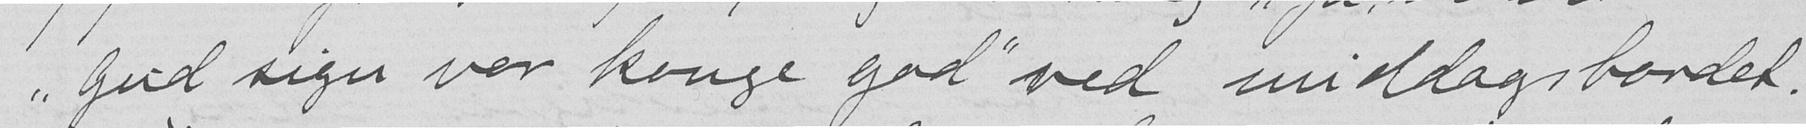og "Gud sign vor konge god" ved middagsbordet.
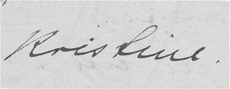Kristine.
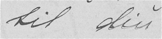til din
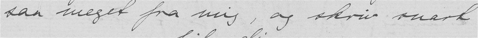saa meget fra mig, og skriv snart
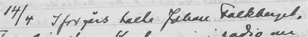17/4 Iforgårs talte Johan Falkberget,
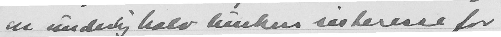en underlig halv brukers interesse for
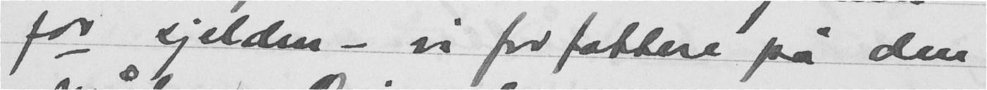for sjelden - vi forfattere på den
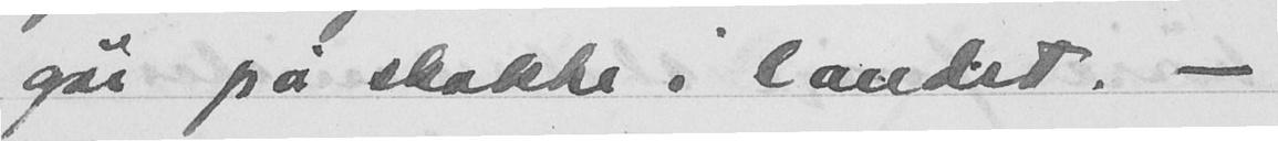går på skakke i landet. -
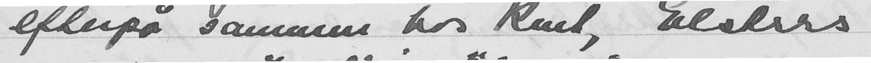efterpå sammen hos Kent, Elsters
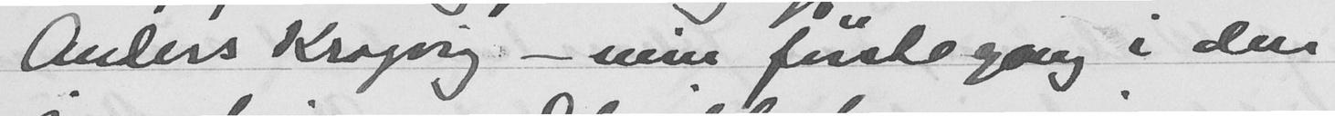Anders Kraprig - min førstegang i den
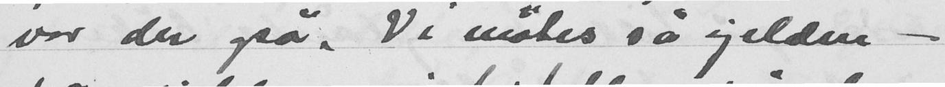var der også. Vi møtes så sjelden -
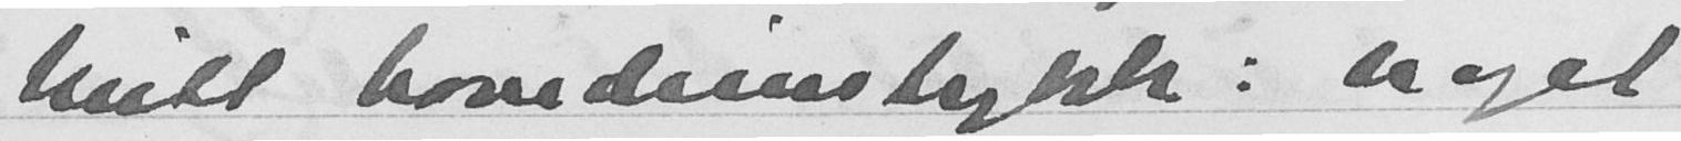Mitt hovedinntrykk: noget
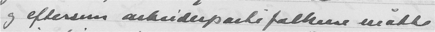og eftersom arbeiderpartifolkene måtte
